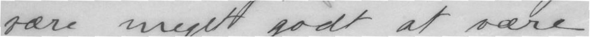være meget godt at være
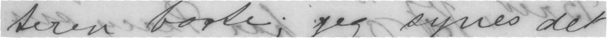teren borte; jeg synes det
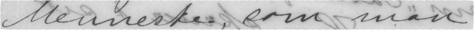Menneske, som man
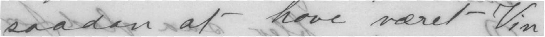saadan at have været Vin
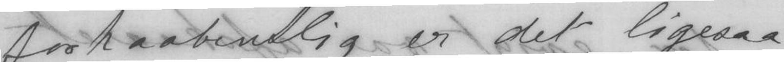forhaabentlig er det ligesaa
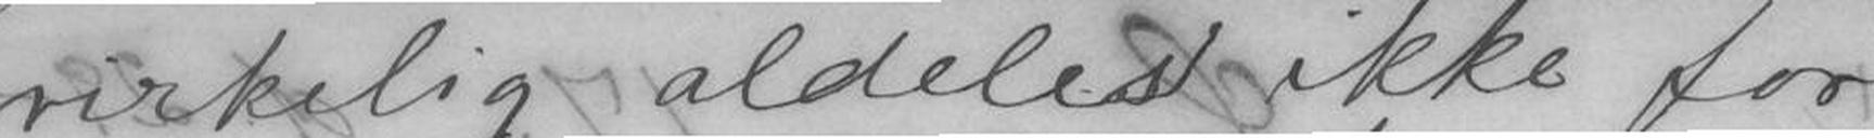virkelig aldeles ikke for
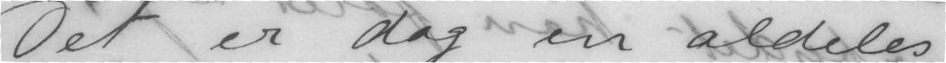Det er dog en aldeles
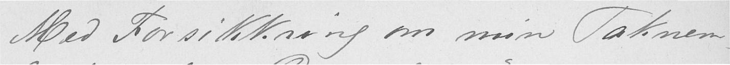Med Forsikkring om min Taknem-
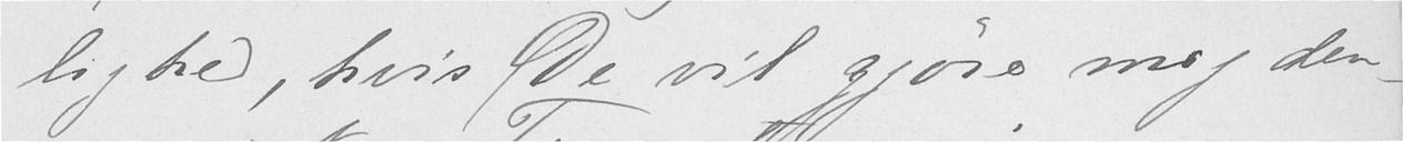lighed, hvis De vil gjøre mig den-
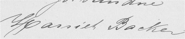Harriet Backer
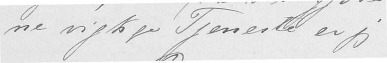ne vigtige Tjeneste er jeg
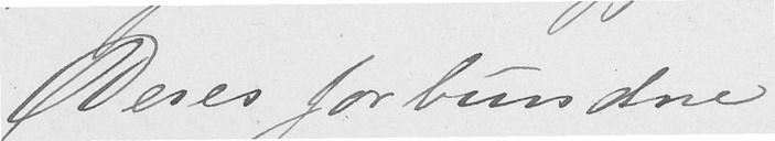Deres forbundne
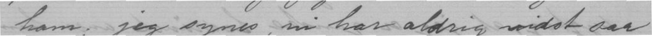ham; jeg synes, vi har aldrig vidst saa
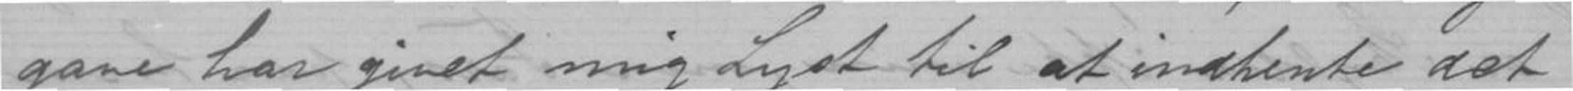gave har givet mig Lyst til at indhendte det
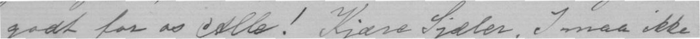godt for os Alle! Kjære Skjæler, I maa ikke
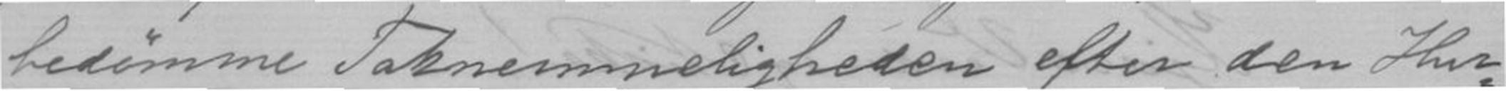bedømme Taknemmeligheden efter den Hur
